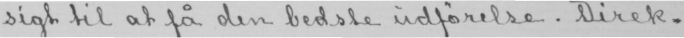sigt til at få den bedste udførelse. Direk-
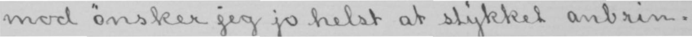mod ønsker jeg jo helst at stykket anbrin-
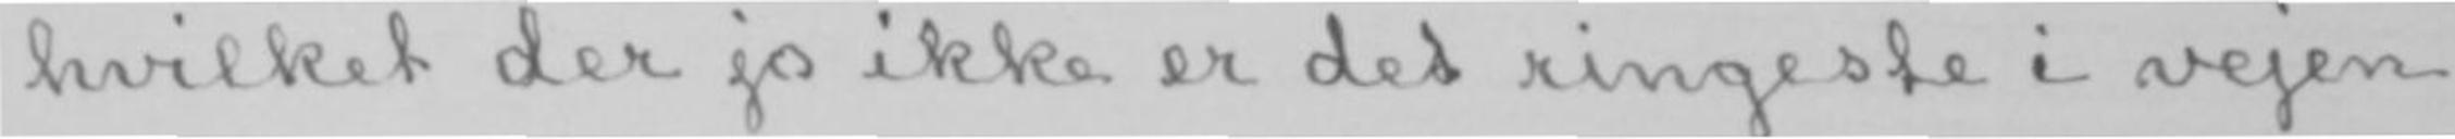hvilket der jo ikke er det ringeste i vejen
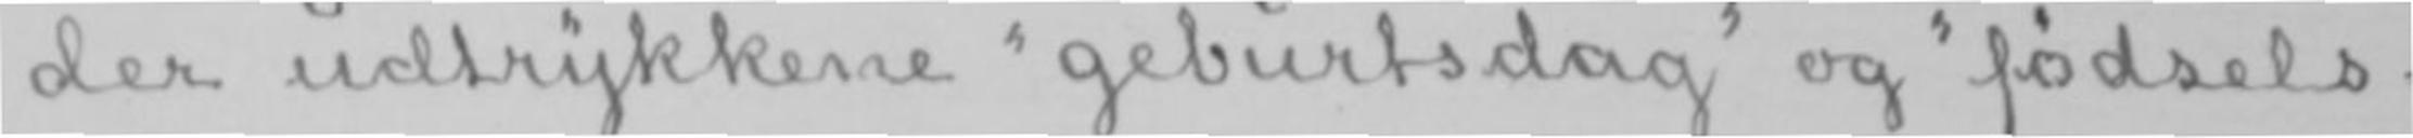der udtrykkene «geburtsdag» og «fødsels-
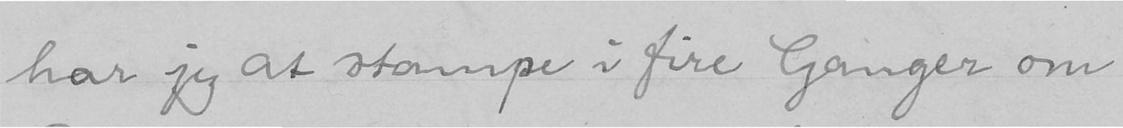har jeg at stampe i fire Ganger om
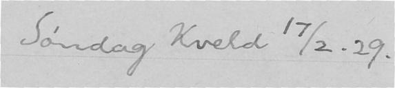Søndag Kveld 17/2. 29.
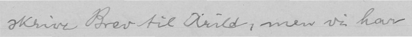skrive Brev til Arild, men vi har
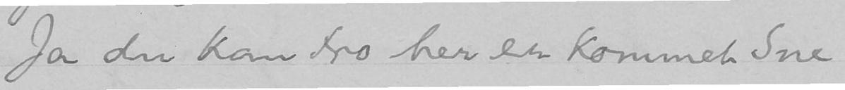Ja du kan tro her er kommet Sne
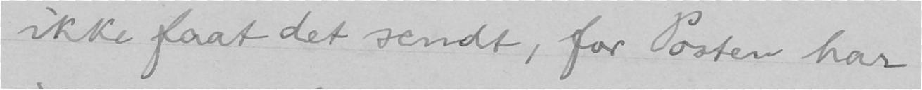ikke faat det sendt, for Posten har
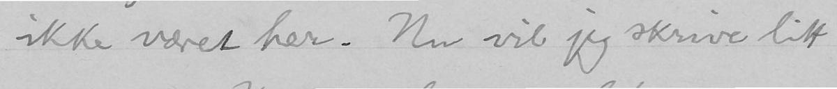ikke været her. Nu vil jeg skrive litt
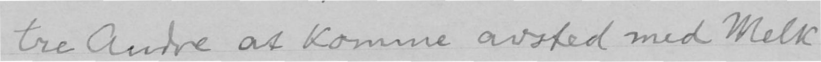tre Andre at komme avsted med Melk
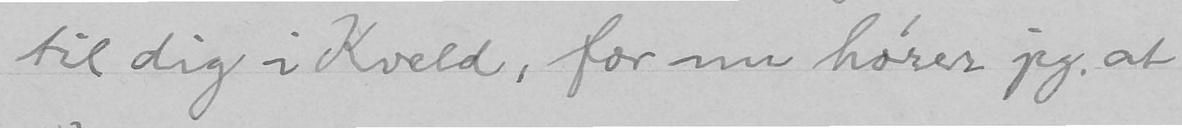til dig i Kveld, for nu hører jeg at
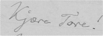Kjære Tore!
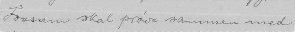Fossum skal prøve sammen med
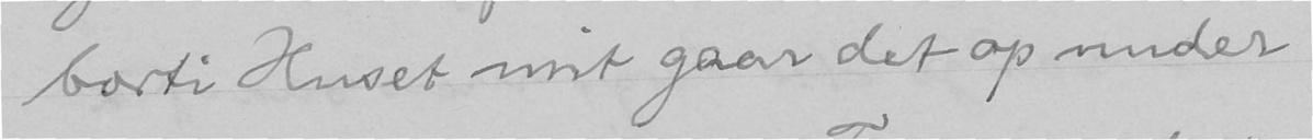borti Huset mit gaar det op under
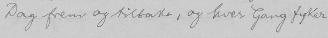Dag frem og tilbake, og hver Gang fyker
